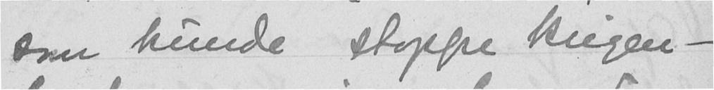som kunde stoppe krigen -
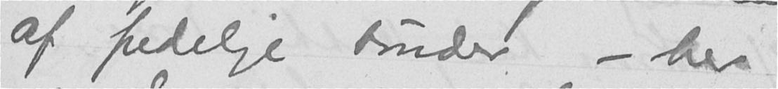af fredelige bønder - her
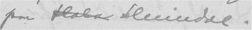paa Hola Heimdal.
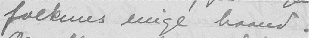folkenes evige haand.
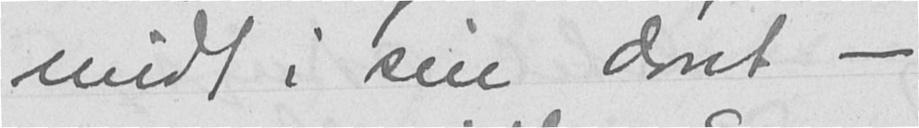midt i sin dont -
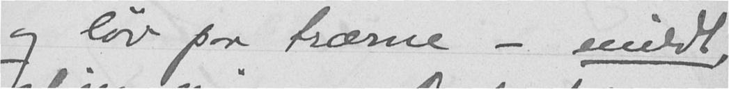og løv paa trærne - mildt,
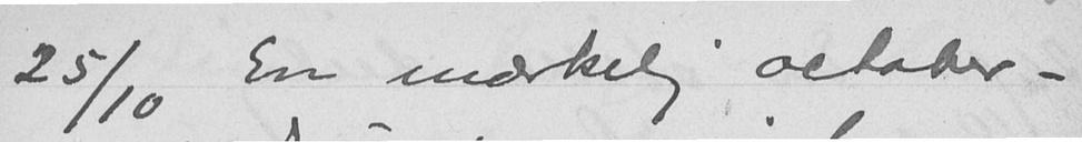25/10 En mærkelig october -
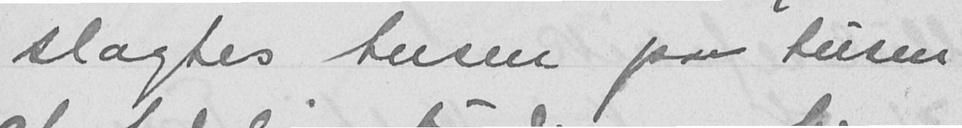slagtes tusen paa tusen
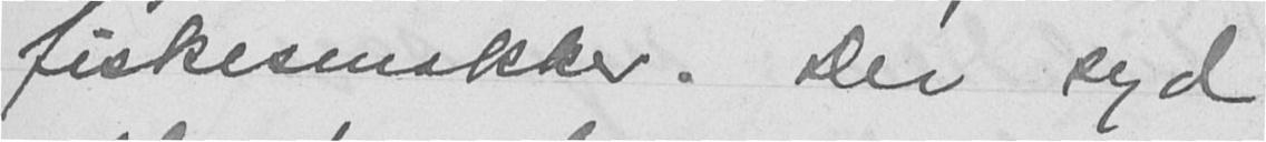fiskesmakker. Der syd
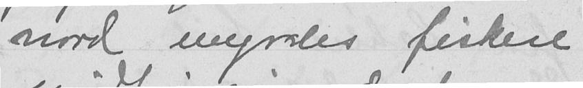nord myrdes fiskere
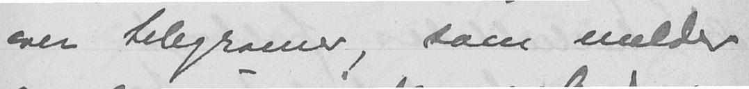over telegramer, som melder
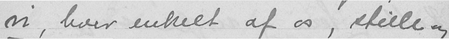vi, hver enkelt af os, stille og
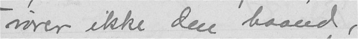rører ikke den haand,
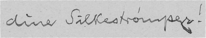dine Silkestrømper!
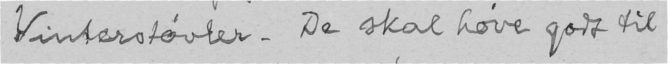Vinterstøvler. De skal høve godt til
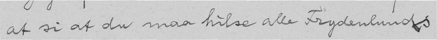at si at du maa hilse alle Frydenlunds
